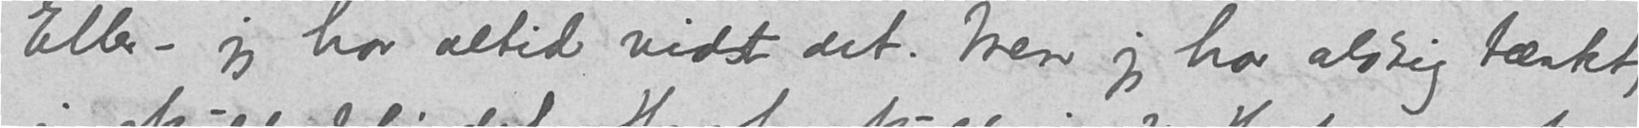Eller — jeg har altid vidst det. Men jeg har aldrig tænkt,
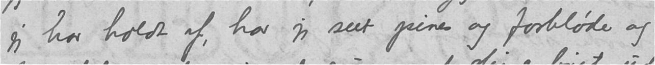jeg har holdt af, har jeg seet pines og forbløde og
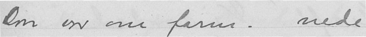Don var om form. nede
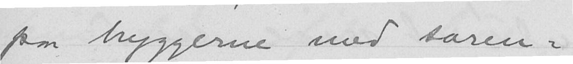paa bryggerne med soren-
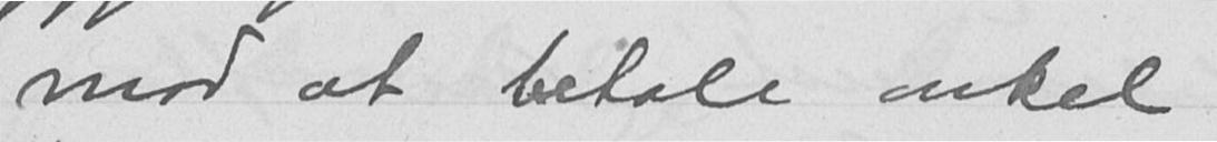mod at betale onkel
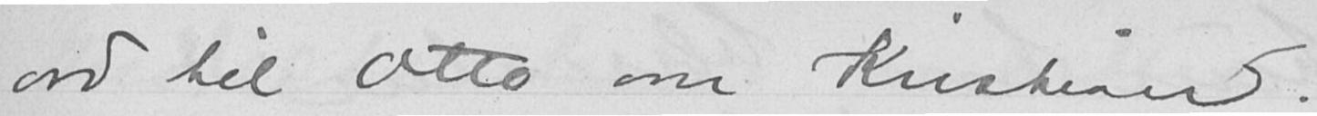ord til Otto om Kristian.
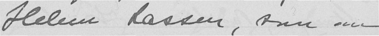Helene Lassen, som om
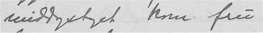middagstoget kom fru
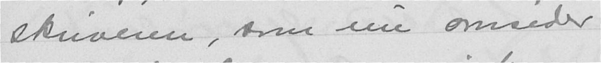skriveren, som nu omsider
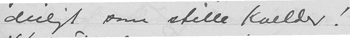deiligt som stille kvelder!
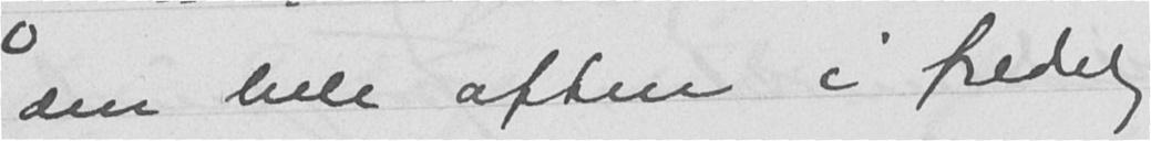den hele aften i fredelig
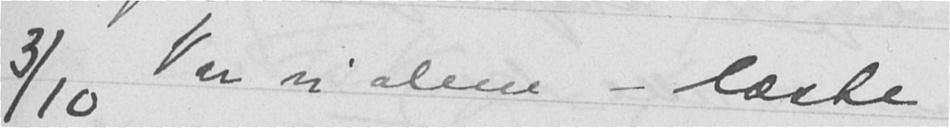3/10 Var vi alene - læste
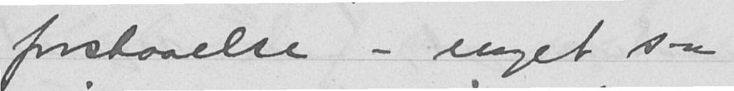forstaaelse - noget saa
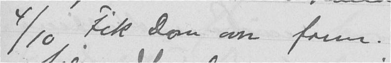4/10 Fik Don om form.
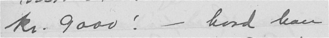kr. 9000! - hvad han
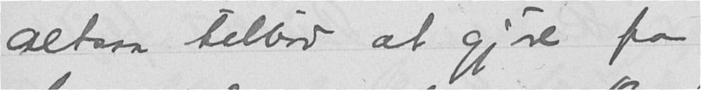altsaa tilbød at gjøre fra
